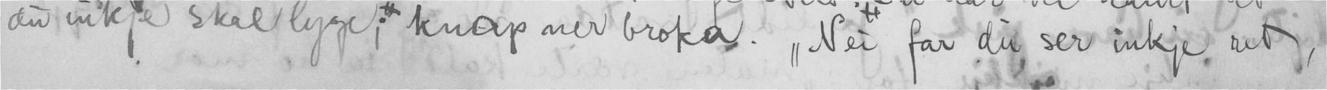en inkje skal lyge; knap ner broka. "Nei far du ser inkje ret,
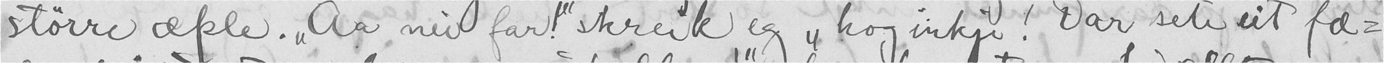større æple. "Aa nu far!" skreik eg "hog inkje! Dar sete eit fæ-
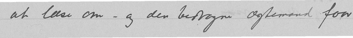at læse om - og den bedragne ægtemand faar
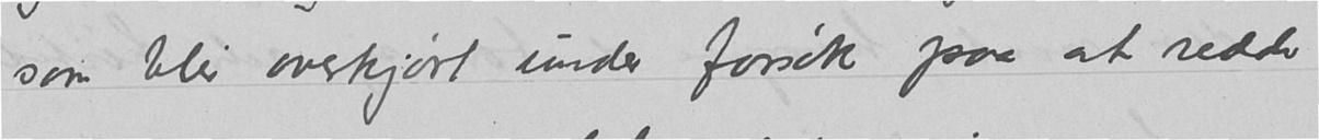som blir overkjørt under forsøk paa at redde
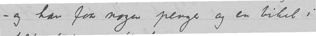– og han faar nogen penger og en bibel i
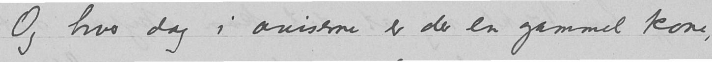Og hver dag i aviserne er der en gammel kone,
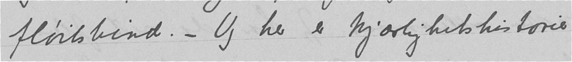fløilsbind. - Og her er kjærlighetshistorier
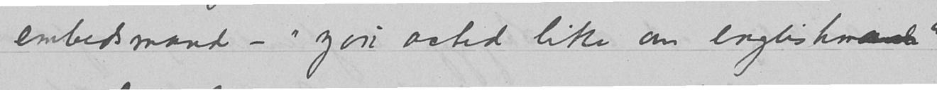embedsmand -«you acted like an englishman»
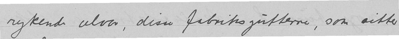rykende alvor, disse fabriksgutterne, som sitter
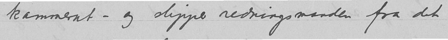kammerat - og slipper redningsmanden fra det
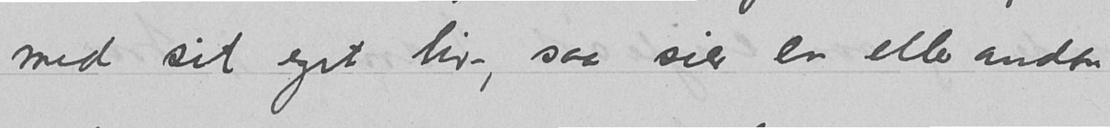med sit eget liv, saa sier en eller anden
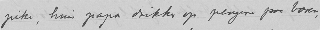pike, hvis papa drikker op pengene paa baren,
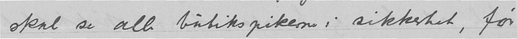skal se alle butikspikerne i sikkerhet, før
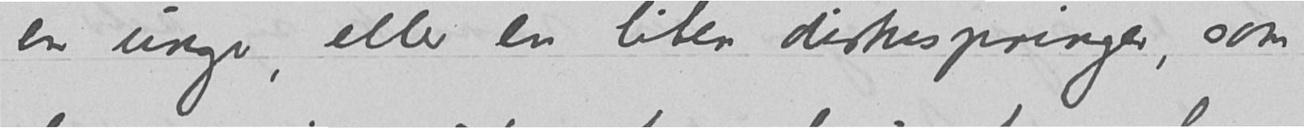en unge, eller en liten diskespringer, som
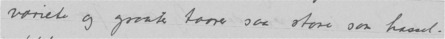variete og graater taarer saa store som hassel-
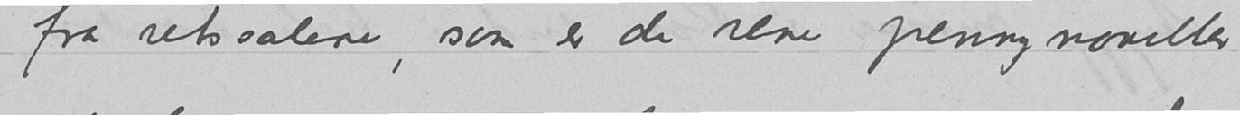fra retssalene, som er de rene pennynoveller
